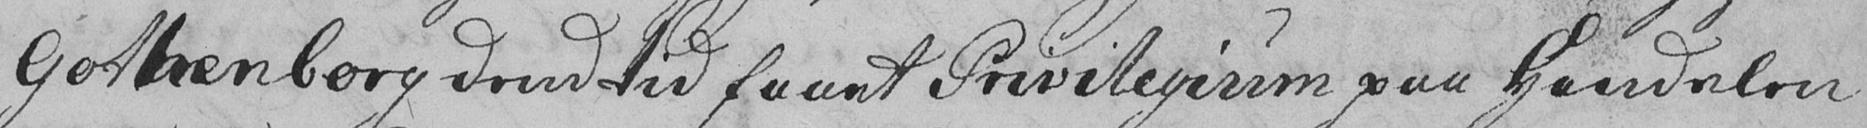Gothenborg dend tid faaet Privilegium paa Handelen
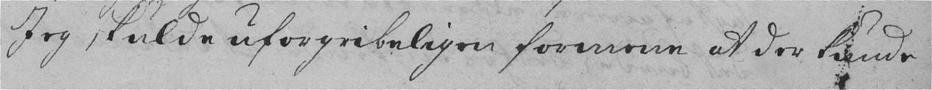Ieg skulde uforgribeligen formene at der kunde
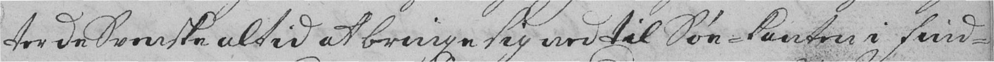ter de Svenske altid at bringe sig ned til Søe-Kanten i Find-
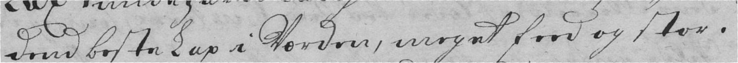den beste Lax i Værden, meget feed og stor.
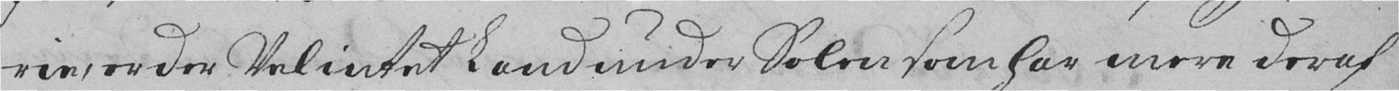rie, er der vel intet Land under Solen som har mere deraf
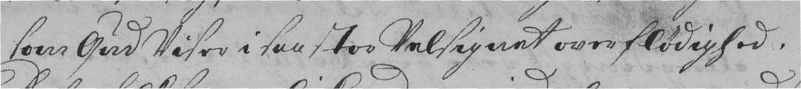som Gud Viser i saa stor Velsignet overflødighed.
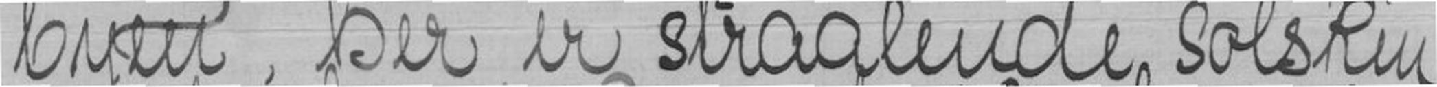byen, her er straalende solskin
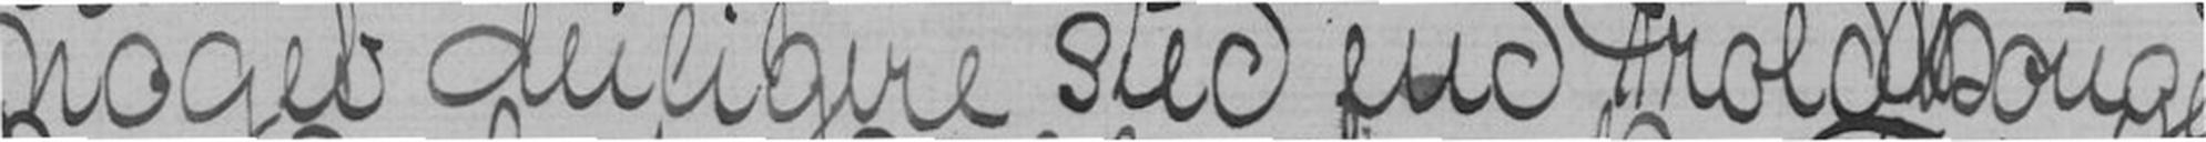Noget deiligere sted end Troldhougen
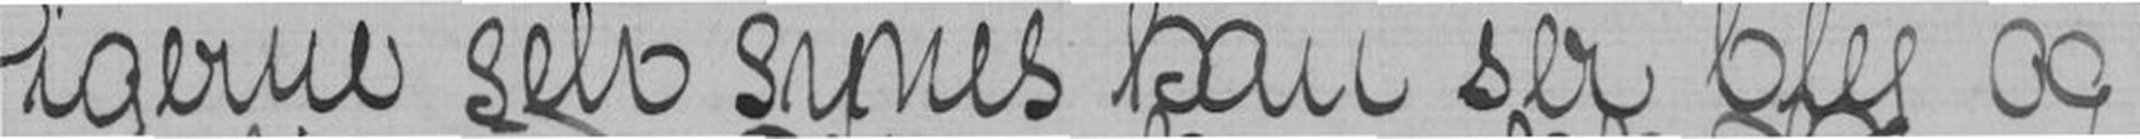Pigerne selv synes han ser bleg og
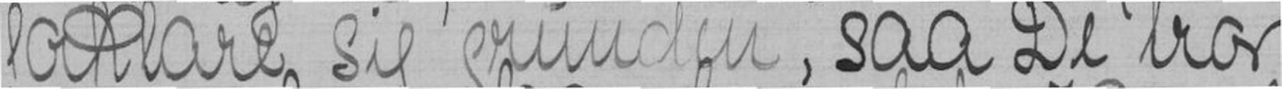forklare sig grunden, saa De tror
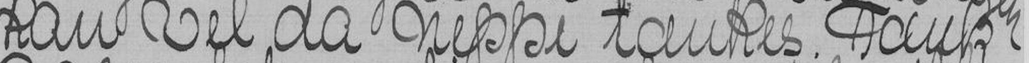kan vel da neppe tænkes. Tante
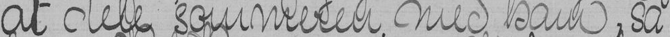at dele sommeren med ham, så
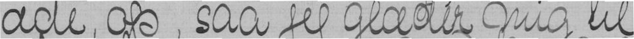æde op, saa jeg glæder mig til
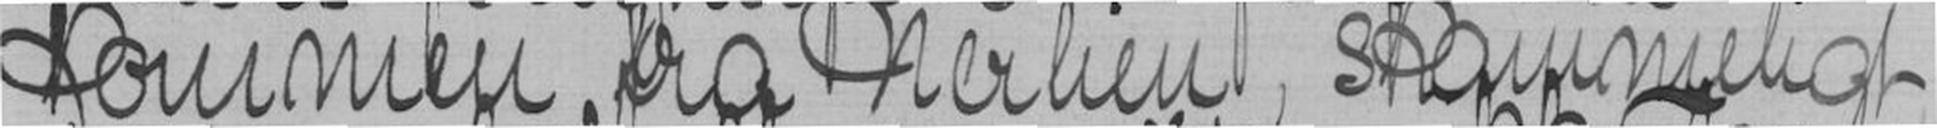kommen fra Nerlien, skammeligt,
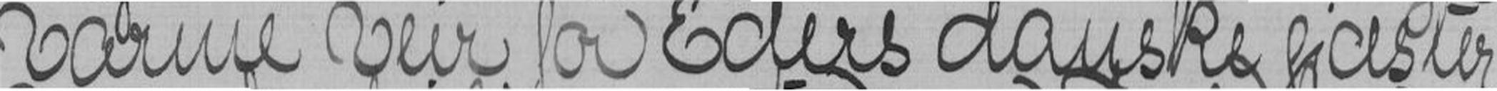varme veir for Eders danske gjæster.
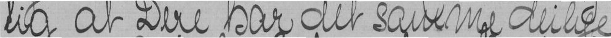tig at Dere har det samme deilige
